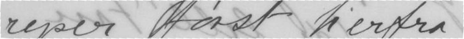rejser først herfra
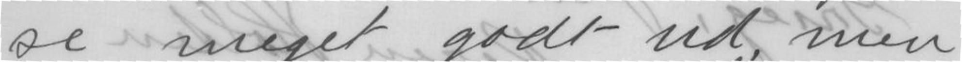se meget godt ud, men
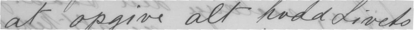at opgive alt hvad Livets
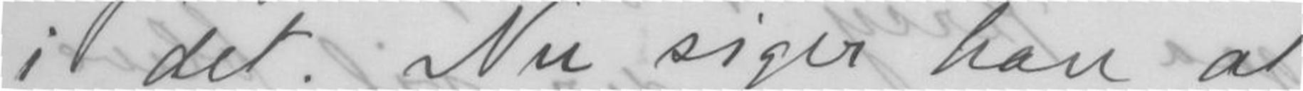i det. Nu siger han at
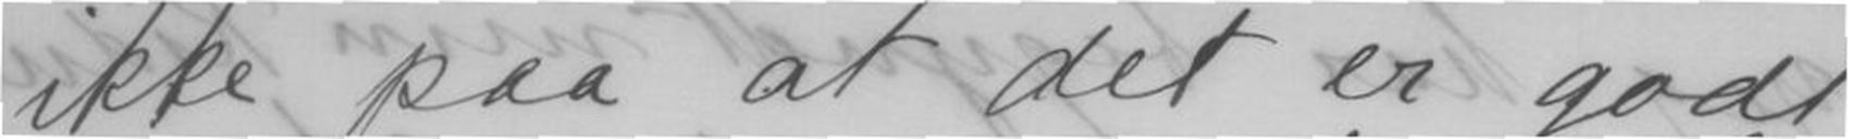ikke paa at det er godt
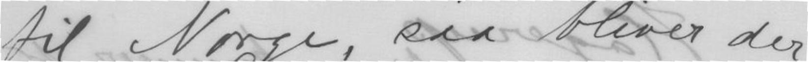til Norge, saa bliver der
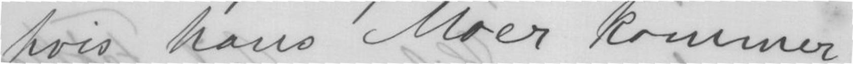hvis hans Moer kommer
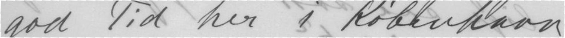god Tid her i København,
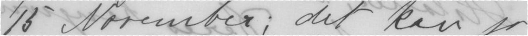15 November; det kan jo
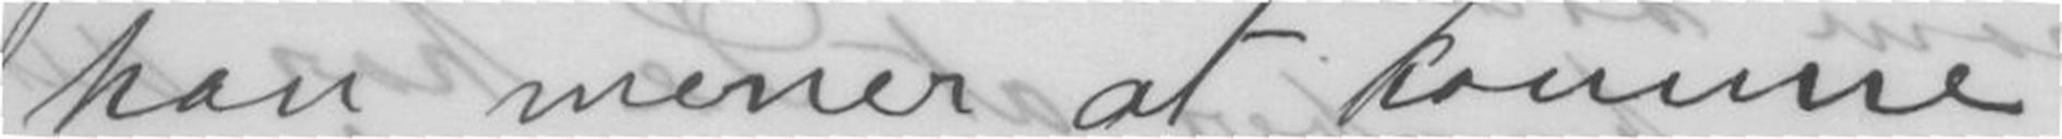han mener at komme
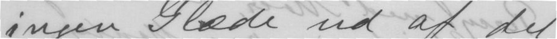ingen Glæde ud af det
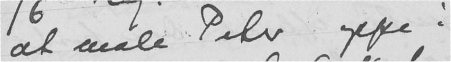at male Peter oppe i
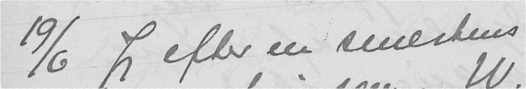19/6 Jeg efter en smertens
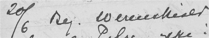20/6 Beg. Werenskiold
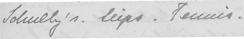Schultz's. Seips. Tennis.
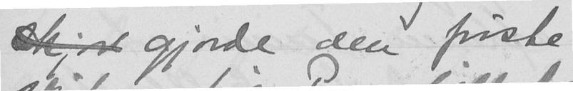skjød gjorde den første
